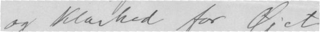og Klarhed for Øjet.
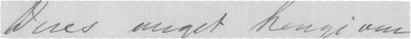Deres meget Hengivne
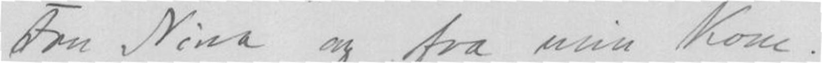Fru Nina og fra min Kone.
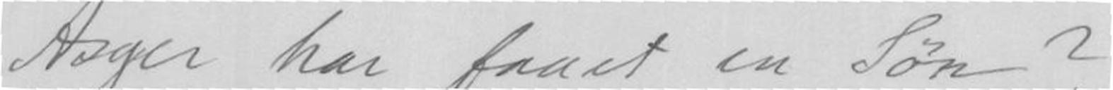Asger har faaet en Søn?
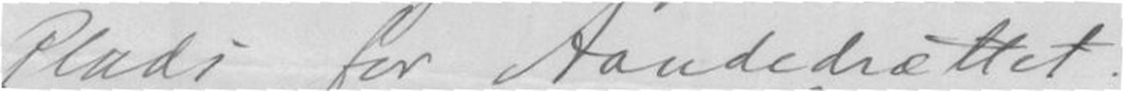Plads for Aandedrættet
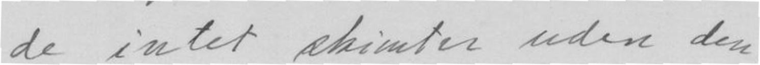de intet skimter uden den.
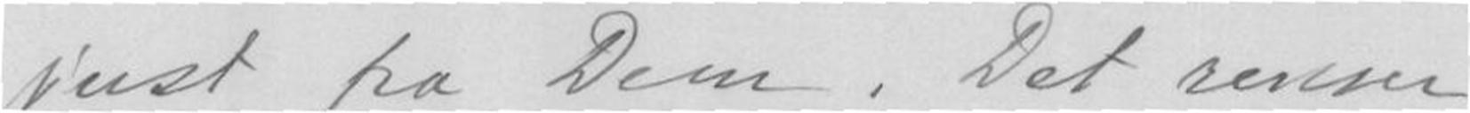just fra Dem. Det renser
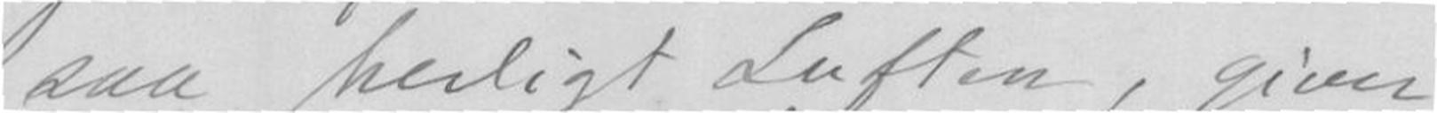saa herligt Luften, giver
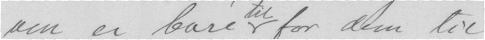ven er bare til for dem til
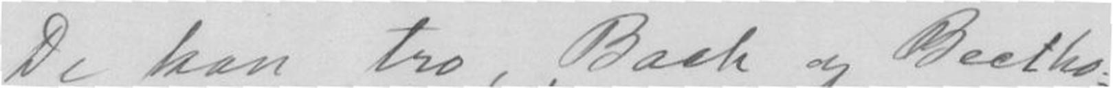De kan tro, Bach og Beetho-
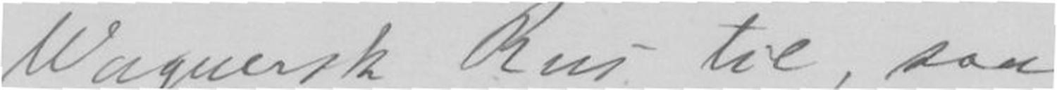Wagnersk Rus til, saa
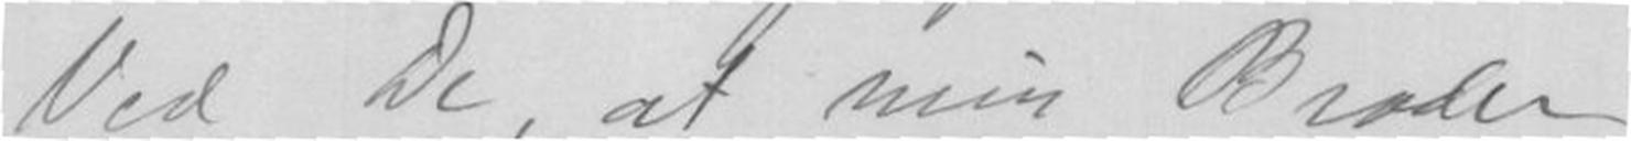Ved De, at min Broder
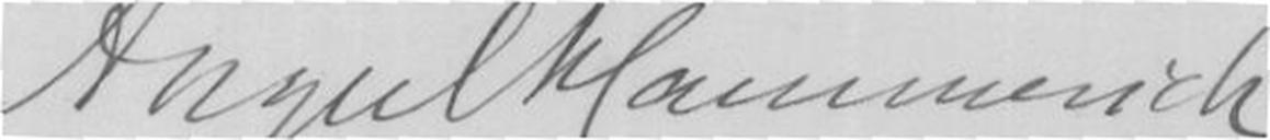Angul Hammerich
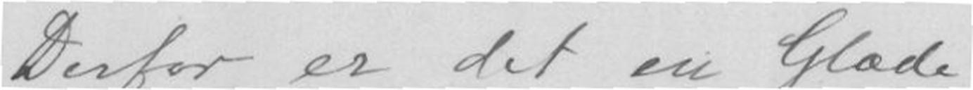Derfor er det en Glæde,
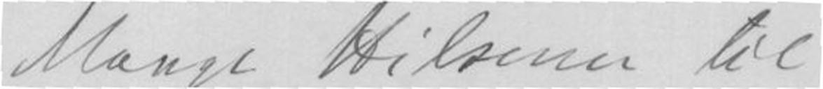Mange Hilsener til
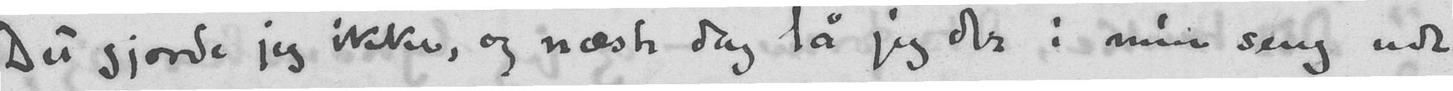Det gjorde jeg ikke, og næste dag lå jeg der i min seng ude
